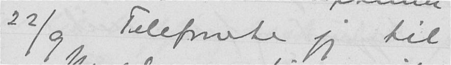22/9 Telefonerte jeg til
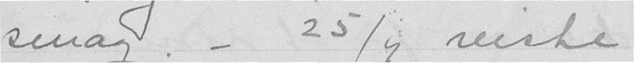smag. - 25/9 reiste
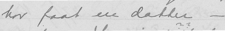har faat en datter -
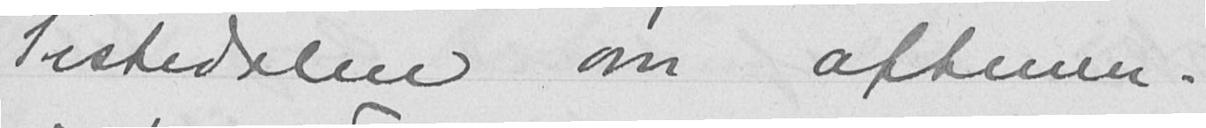Tistedalen om aftenen.
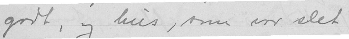godt, og hus, som var slet
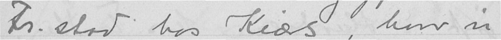Fr. stad hos Kiærs, hvor vi
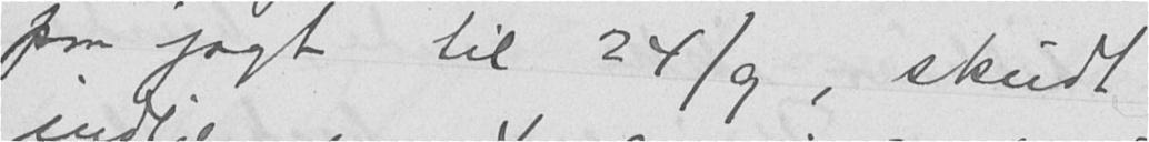paa jagt til 24/9, skudt
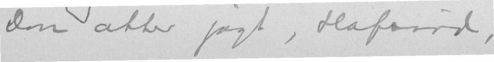Don atter jagt, Hafsrød,
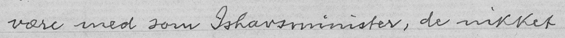være med som Ishavsminister, de nikket
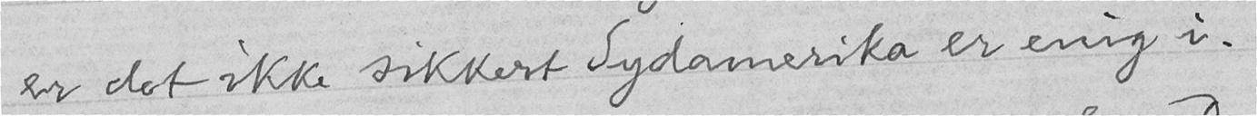er det ikke sikkert Sydamerika er enig i.
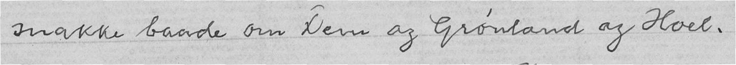snakke baade om Dem og Grønland og Hoel.
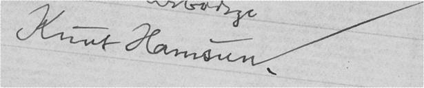Knut Hamsun.
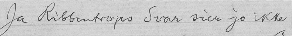Ja Ribbentrops Svar sier jo ikke
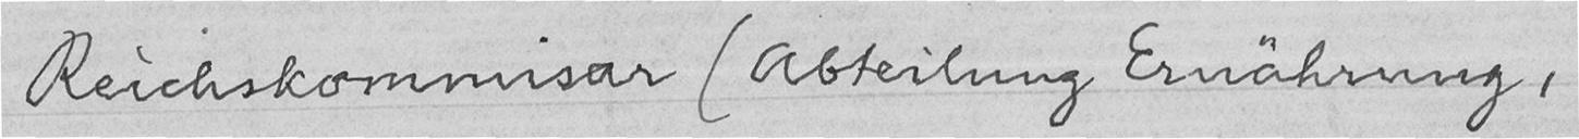Reichskommisar (Abteilung Ernährung,
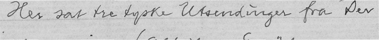Her sat tre tyske Utsendinger fra Der
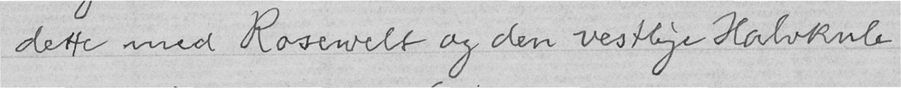dette med Rosewelt og den vestlige Halvkule
